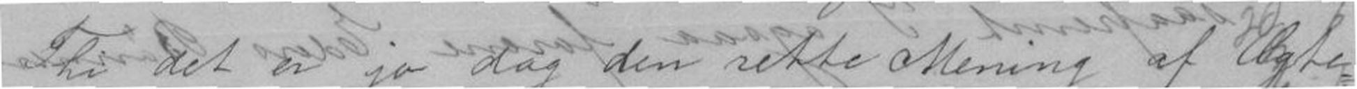Thi det er jo dog den rette Mening af Egte-
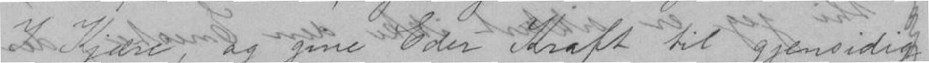I Kjære, og give Eder Kraft til gjensidif
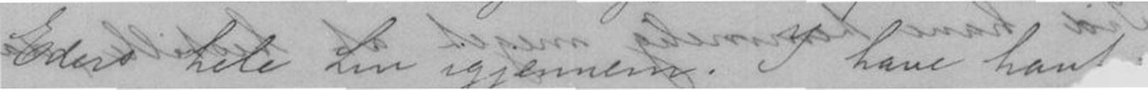Eders hele Liv igjennem. I have havt
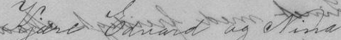Kjære Edvard og Nina!
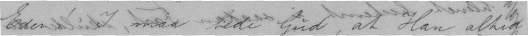Eder! I maa bede Gud, at Han Altid
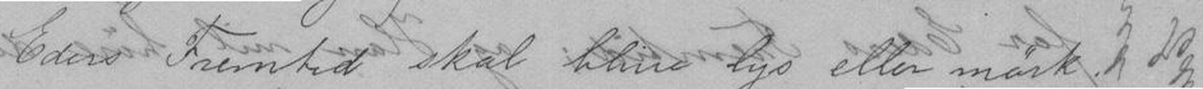Eders Fremtid skal blive lys eller mørk.
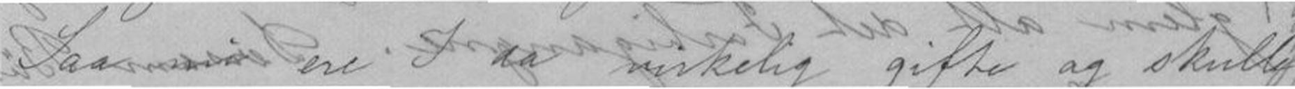Saa nu ere I da virkelig gifte og skulle
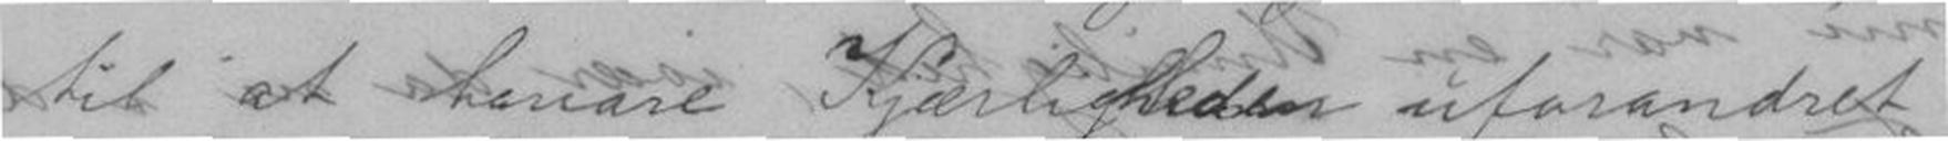til at bevare Kjærligheden uforandret,
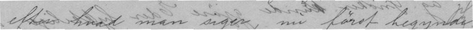efter hvad man siger, nu først begynde
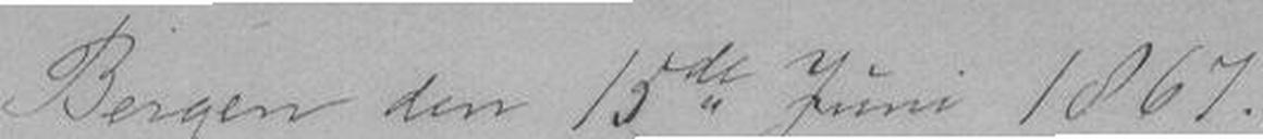Bergen den 15de Juni 1867.
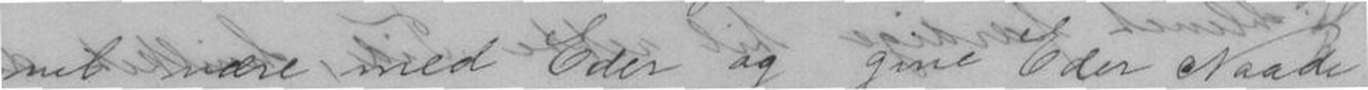vil være med Eder og give Eder Naade
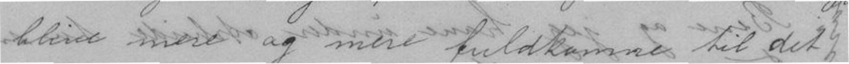blive mere og mere fuldomme til det
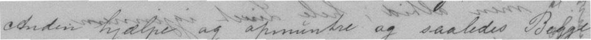Anden hjælpe og opmuntre og saaledes Begge
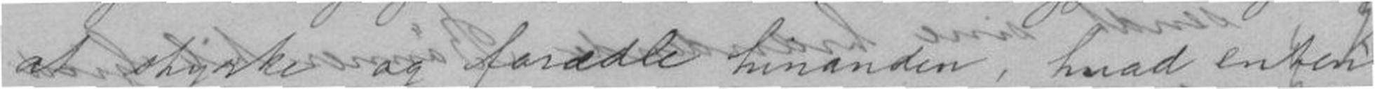at styrke og forædle hinansen, hvad enten
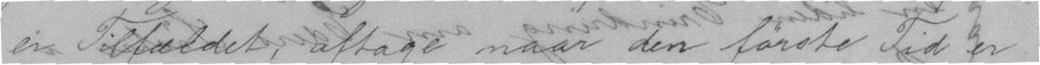er Tilfældet, aftage naar den første Tid er
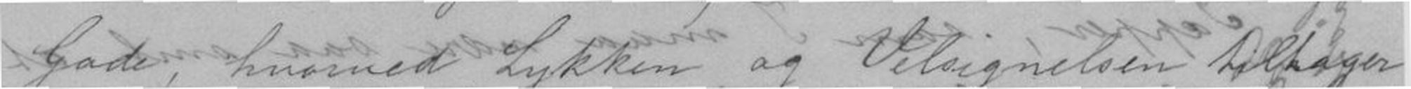Gode, hvorved Lykken og Velsignelsen tiltager
